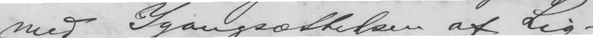med Igangsættelsen af Lig-
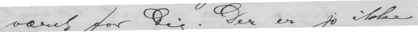været for Dig. Der er jo ikke
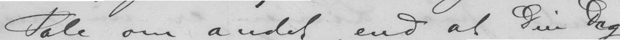Tale om andet, end at Din Dag
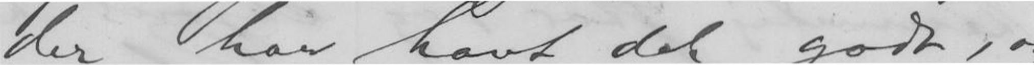der har havt det godt, og
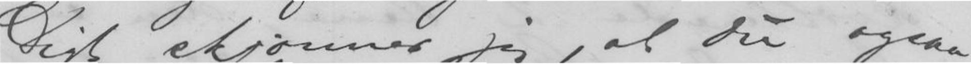Digt skjønner jeg, at du ogsaa
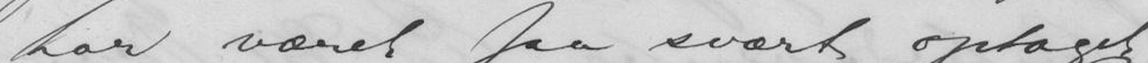har været saa svært optaget
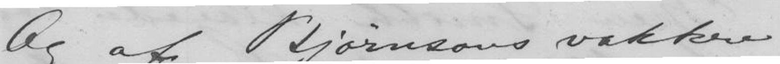Og af Bjørnsons vakkre
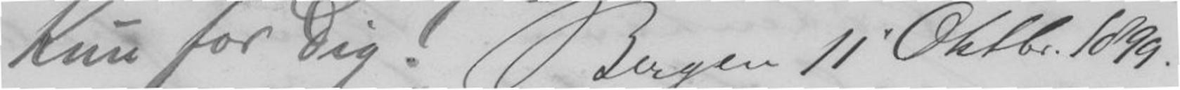Kun for Dig! Bergen 11te Oktober 1899.
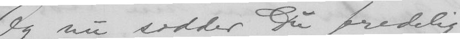Og nu sidder Du fredelig
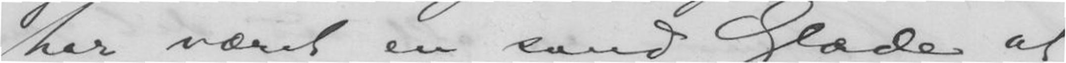har været en sand Glæde at
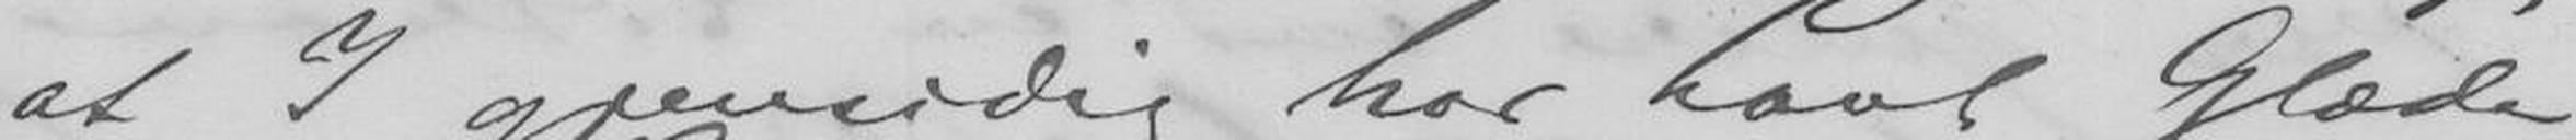at I gjensidig har havt Glæde
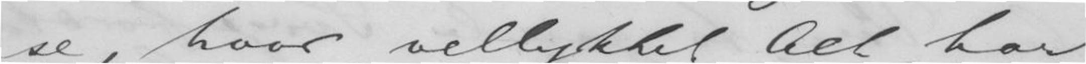se, hvor vellykket Alt har
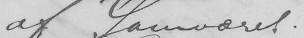af Samværet.
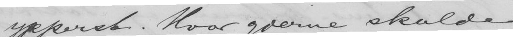ypperste. Hvor gjerne skulde
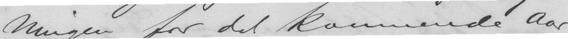ningen for det kommende Aar
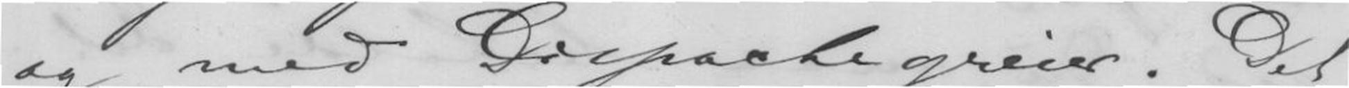og med Dispachegreier. Det
